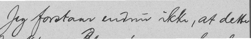Jeg forstaar endnu ikke, at dette
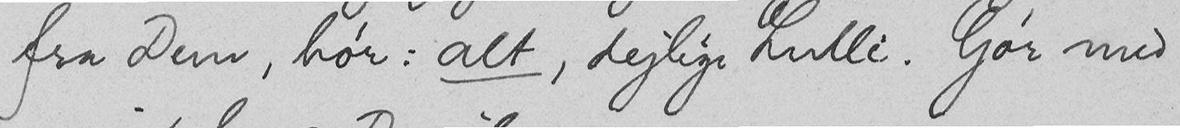fra Dem, hør: alt, dejlige Lulli. Gør med
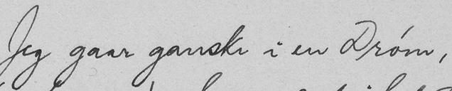Jeg gaar ganske i en Drøm,
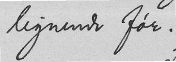lignende før.
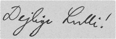Dejlige Lulli!
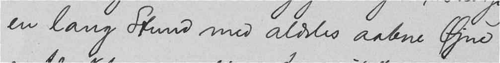en lang Stund med aldeles aabne Øjne
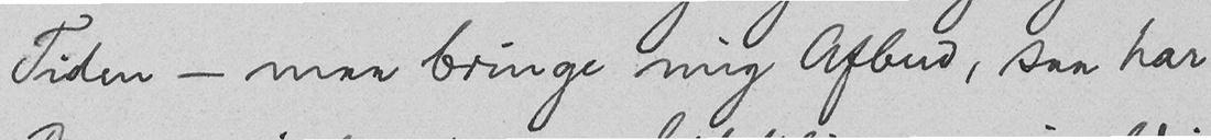Tiden - maa bringe mig Afbud, saa har
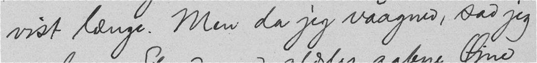vist længe. Men da jeg vaagned, sad jeg
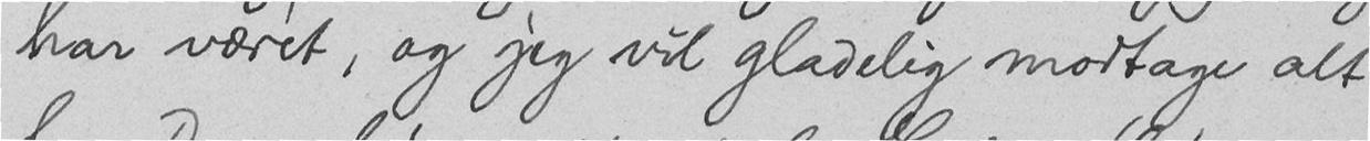har været, og jeg vil gladelig modtage alt
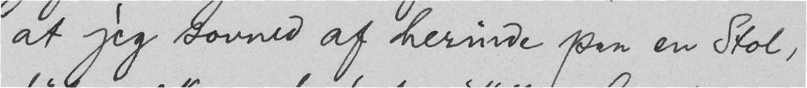at jeg sovned af herinde paa en Stol,
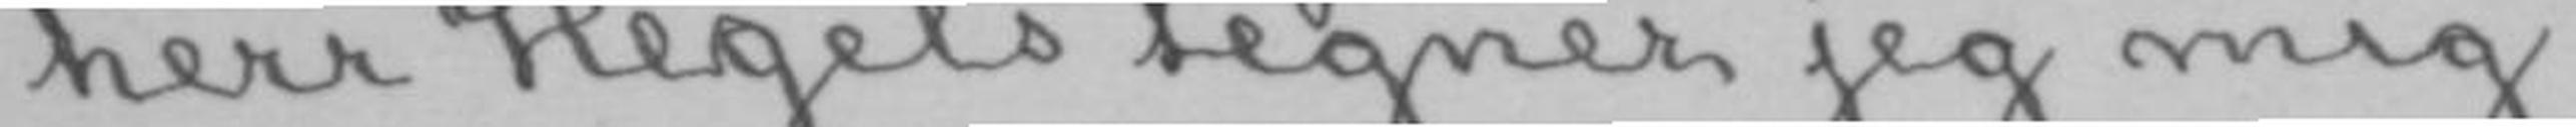herr Hegels tegner jeg mig
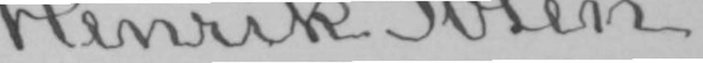Henrik Ibsen.
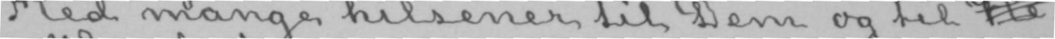Med mange hilsener til Dem og til He
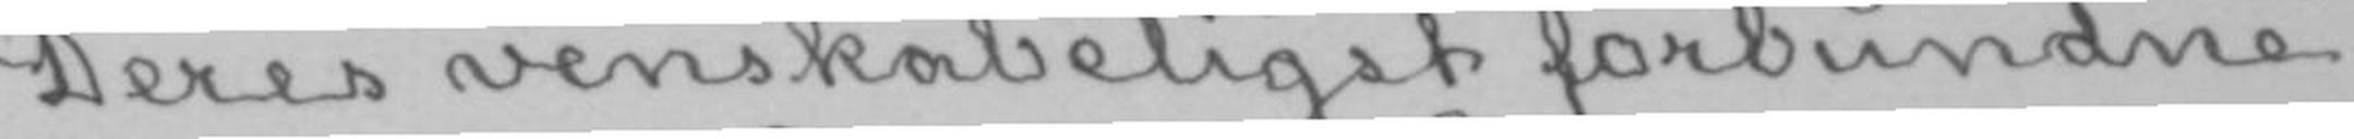Deres venskabeligst forbundne
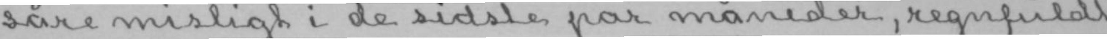såre misligt i de sidste par måneder, regnfuldt
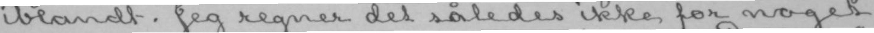iblandt. Jeg regner det således ikke for noget
